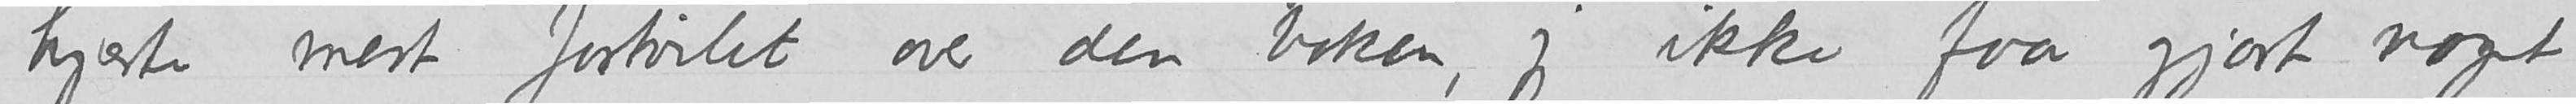hjærte mest fortvilet over den boken, jeg ikke faar gjort noget
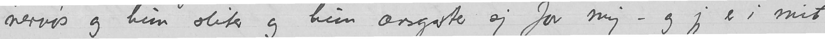nervøs og hun sliter og hun ængster sig for mig – og jeg er i mit
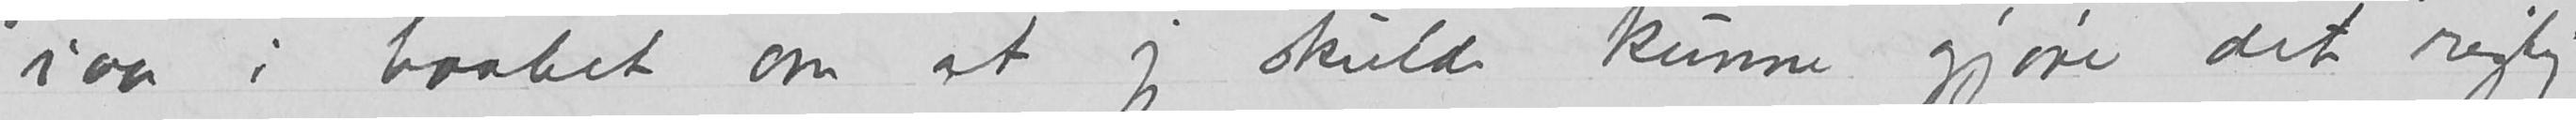iaar i haabet om at jeg skulde kunne gjøre det rigtig
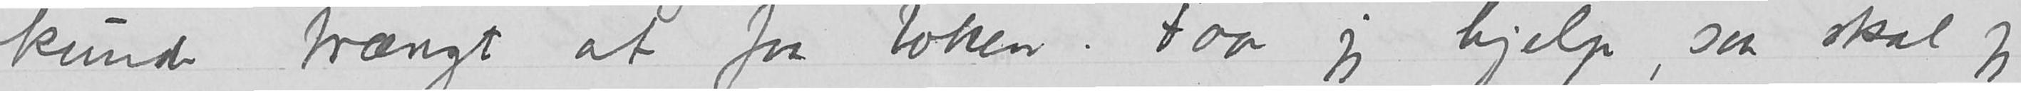kunde trængt at faa boken. Faar jeg hjelp, saa skal jeg
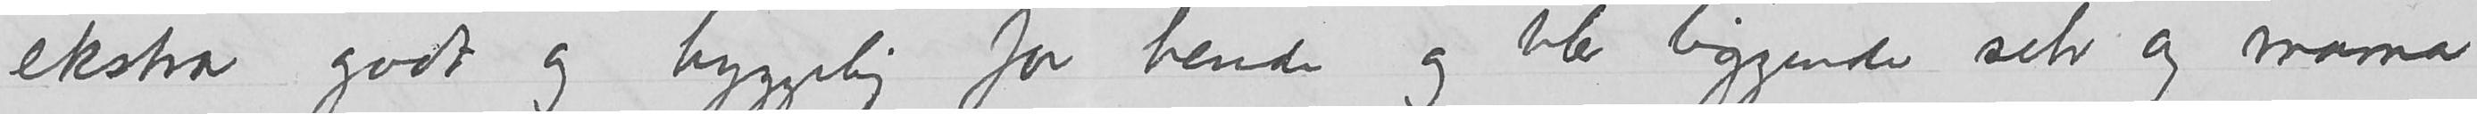ekstra godt og hyggelig for hende og blev liggende selv og mama
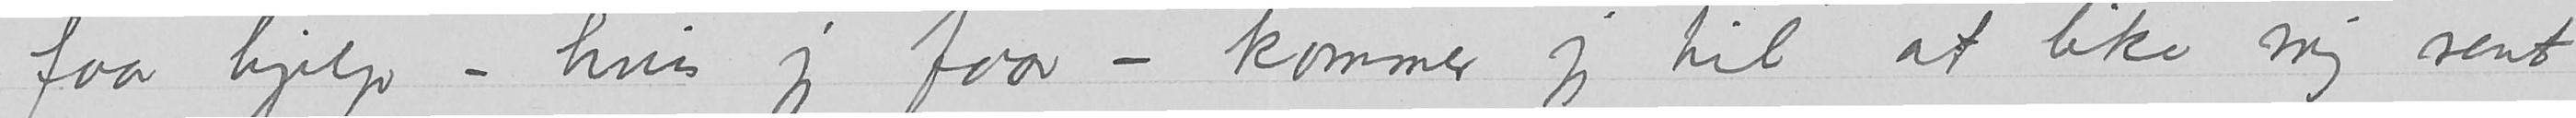faar hjelp – hvis jeg faar – kommer jeg til at like mig rent
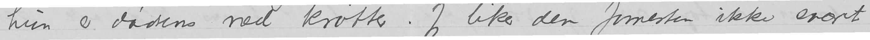hun er dødsens ræd krøtter. Jeg liker dem forresten ikke svært
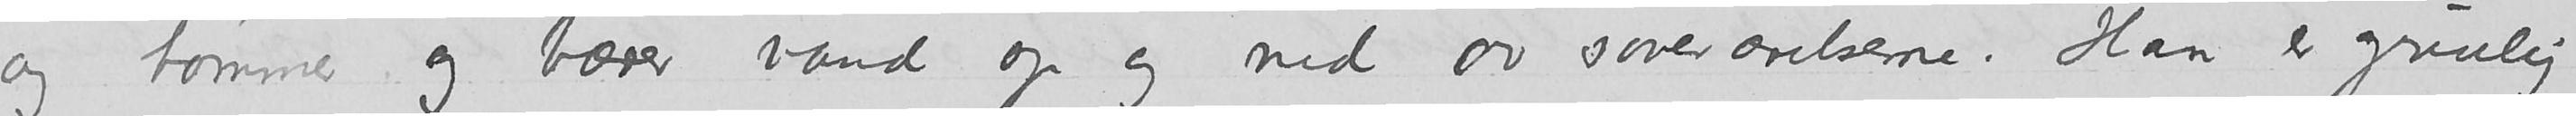og tømmer og bærer vand op og ned av soveværelserne. Han er gruelig
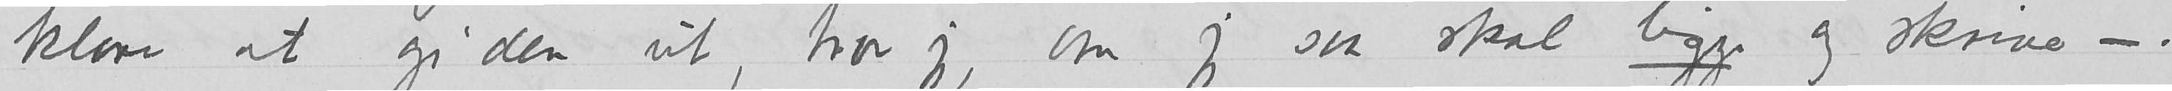klare at gi den ut, tror jeg, om jeg saa skal ligge og skrive –.
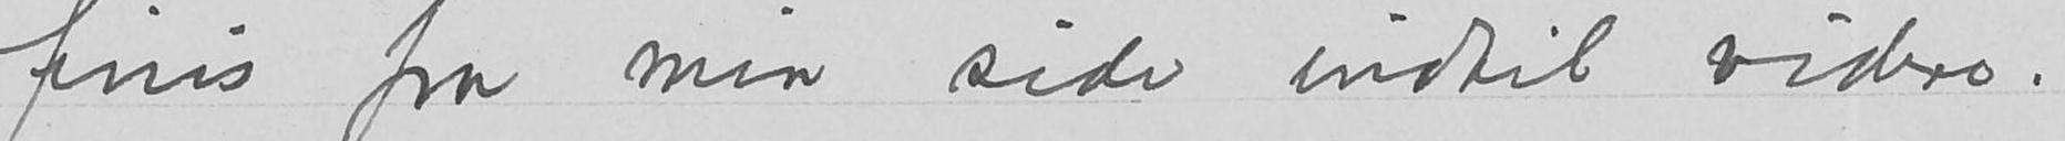finis fra min side indtil videre.
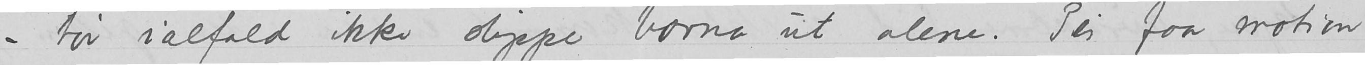– tør ialfald ikke slippe barna ut alene. Pei faar motion
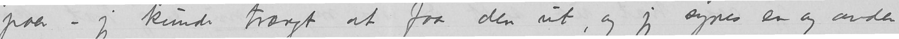paa – jeg kunde trængt at faa den ut, og jeg synes en og anden
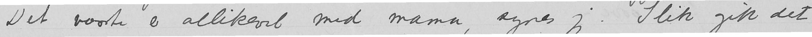Det værste er allikevel med mama, synes jeg. Slik gik det
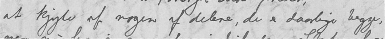at kjyte af nogen af delene, de er daarlige begge,
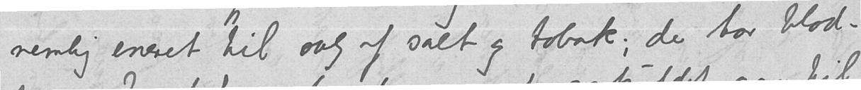nemlig eneret til salg af salt og tobak; de tar blod-
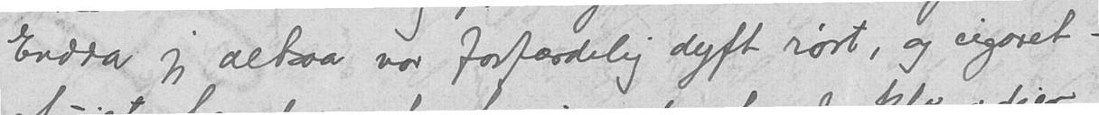Endda jeg altsaa var fortærdelig dypt rørt, og cigaret-
